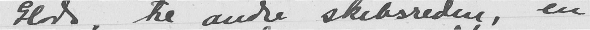Glads, til andre skibsredere, en
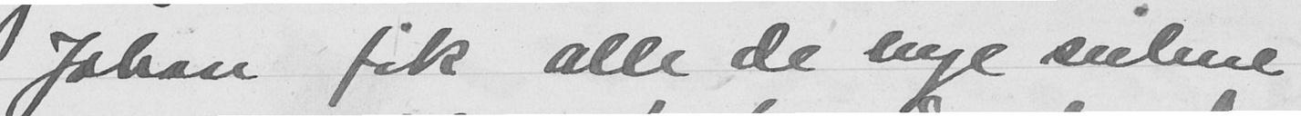Johan fik alle de nye seilene
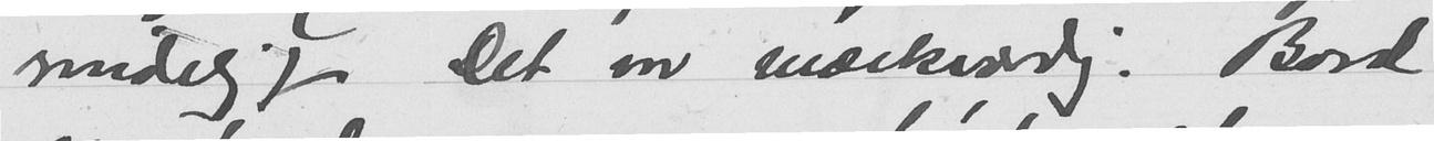rindeligt. Det var mærkverdig. Bord
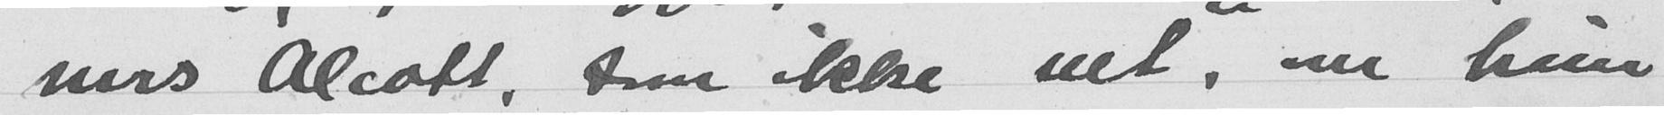mrs Alcott, som ikke vet, om hun
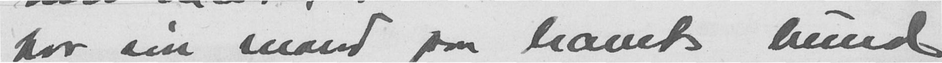har sin mand paa havets bund
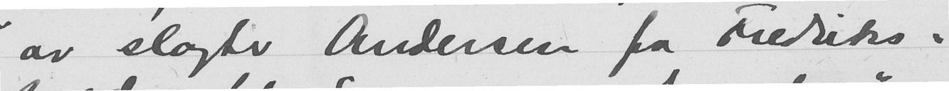av slægte Andersen fra Fredriks-
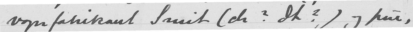vognfabrikant Smit (ch? dt?) og fru,
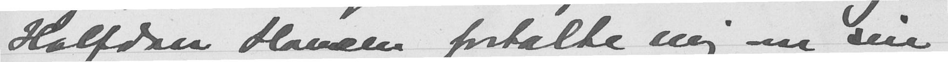Halfdan Hansen fortalte mig om sin
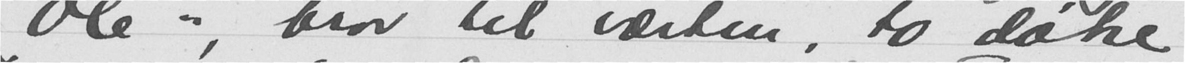"Ole", bror til værten, to dæke
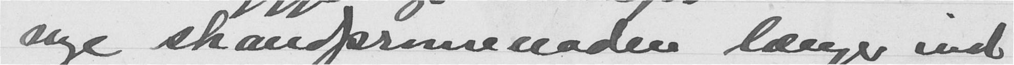nye strandpromenaden længer ind
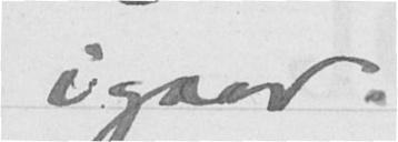igaar.
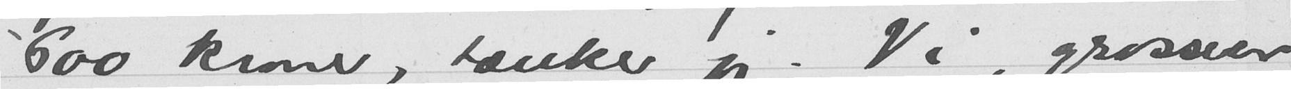600 kroner, tænker jeg. Vi, grosserer
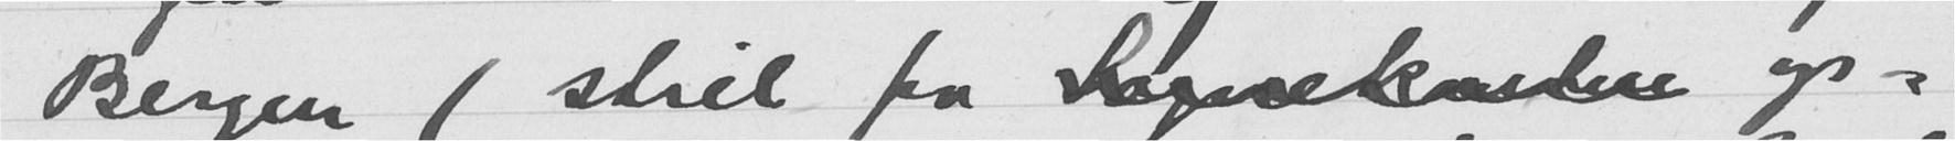Bergen (stril fra Sognekanten op-
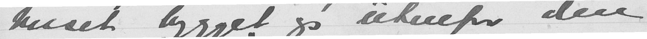huset bygget og utenfor den
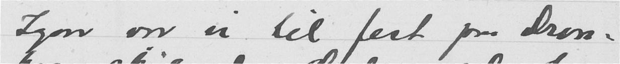Igaar var vi til fest paa Dron-
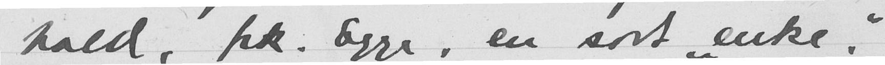hald, frk. Egge, en sort "enke,"
